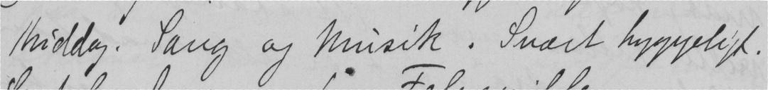Middag. Sang og Musik. Svært hyggeligt.
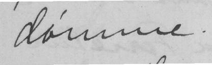dømme.
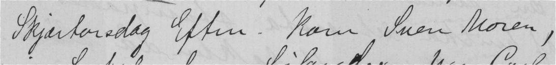Skjærtorsdag Eftm. kom Sven Moren,
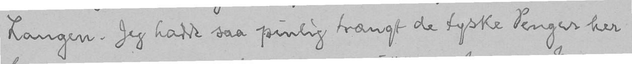Langen. Jeg hadde saa pinlig trængt de tyske Penger her
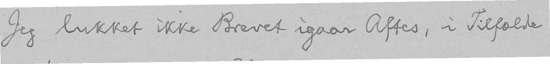Jeg lukket ikke Brevet igaar Aftes, i Tilfælde
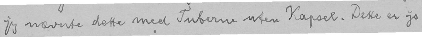jeg nævnte dette med Tuberne uten Kapsel. Dette er jo
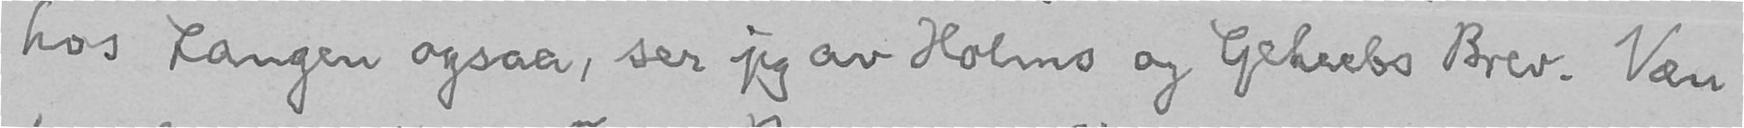hos Langen ogsaa, ser jeg av Holms og Geheebs Brev. Væn
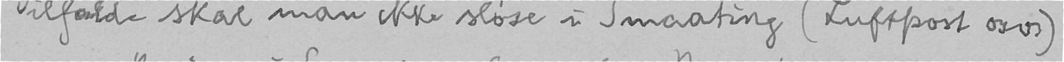Tilfælde skal man ikke sløse i Smaating (Luftpost osv.)
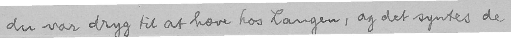du var dryg til at hæve hos Langen, og det syntes de
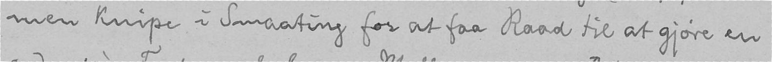men knipe i Smaating for at faa Raad til at gjøre en
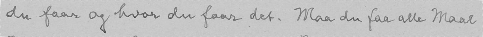du faar og hvor du faar det. Maa du faa alle Maal
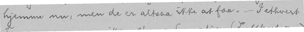hjemme nu, men de er altsaa ikke at faa. - I ethvert
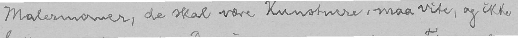Malermaner, de skal være Kunstnere, maa vite, og ikke
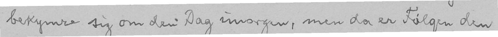bekymre sig om den Dag imorgen, men da er Følgen den
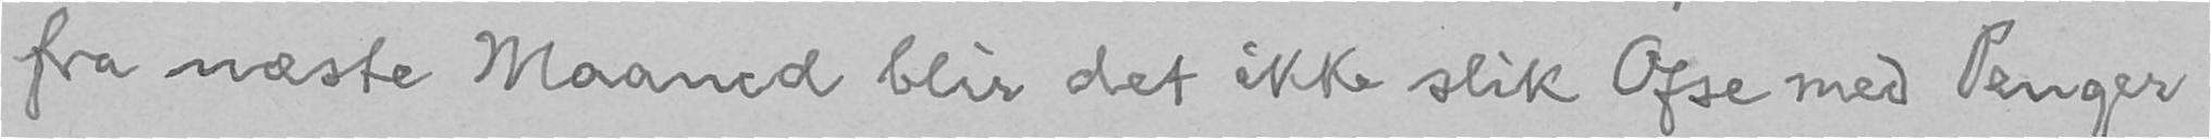fra næste Maaned blir det ikke slik Ofse med Penger
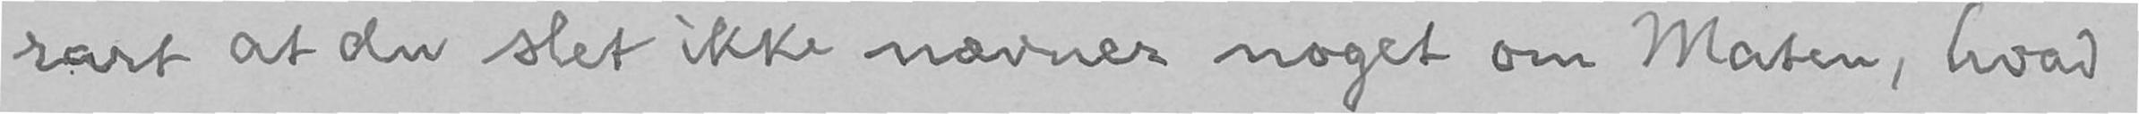rart at du slet ikke nævner noget om Maten, hvad
